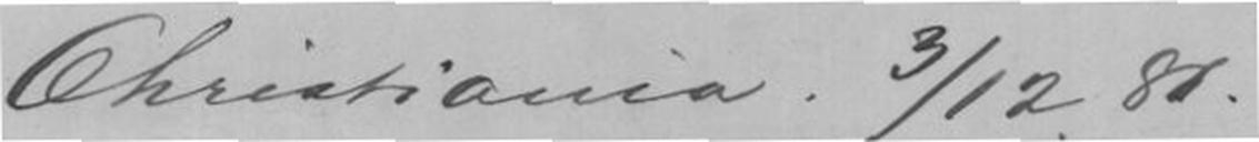Christiania 3/12.81.
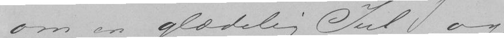om en glædelig Jul og
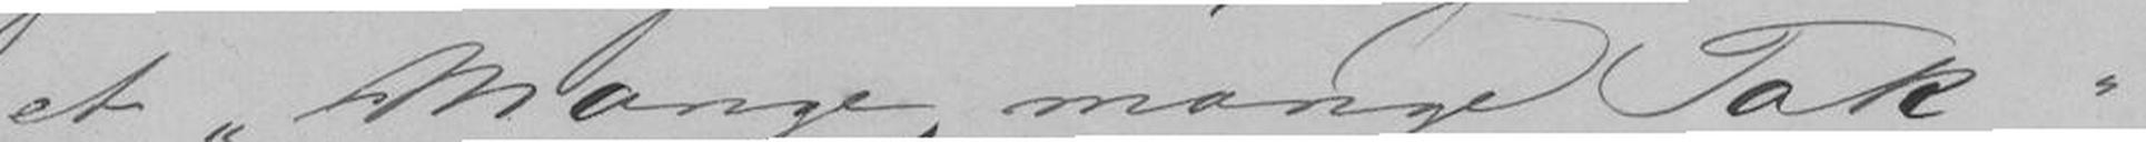et Mange, mange Tak
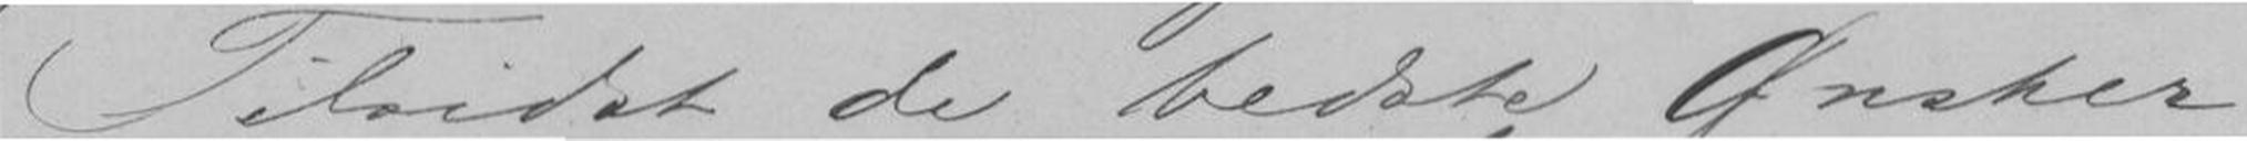Tilsidst de bedste Ønsker
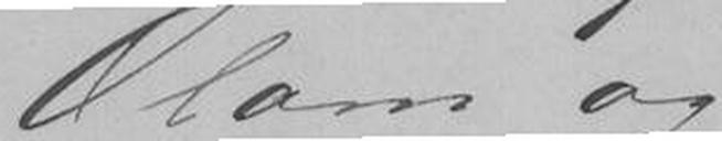Olaus og
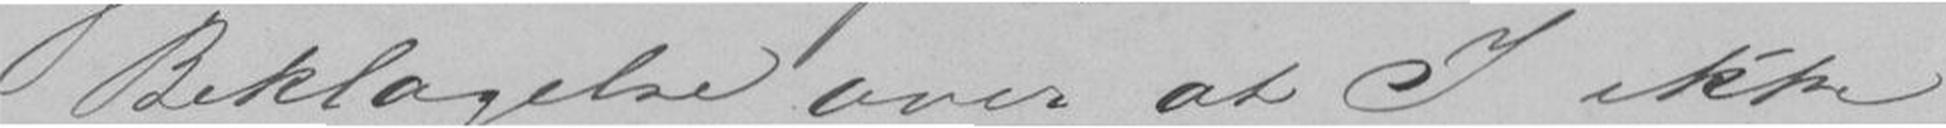Beklagelse over at I ikke
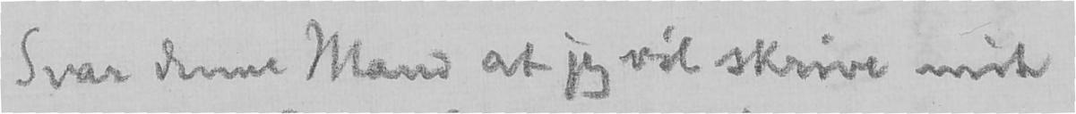Svar denne Mand at jeg vil skrive mit
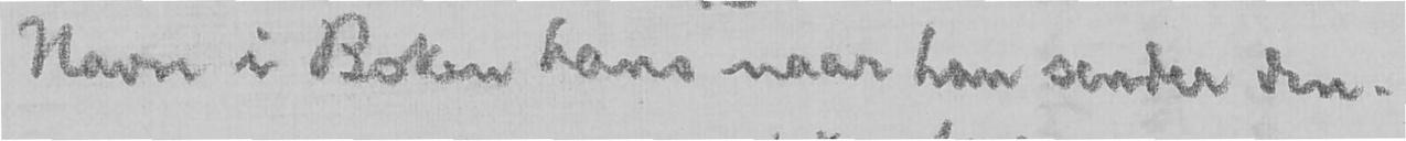Navn i Boken hans naar han sender den.
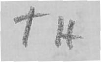TH
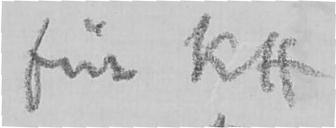für KH
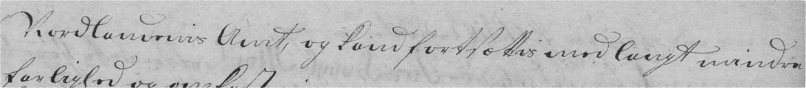Nordlandenis Amt, og kand fortsættis med langt mindre
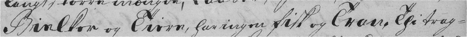Bielker og Tiere, har ingen Fisk og Tran. Thi trag-
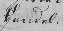Handel.
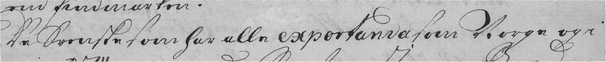De Svenske som har alle exportanda som Norge og i
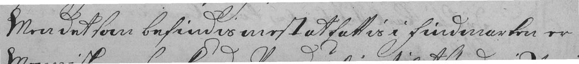Men det som befindis mest at fattis i Findmarken er
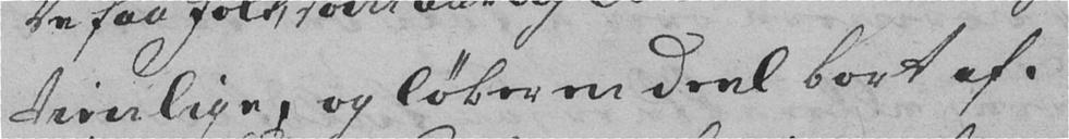trivelige, og løber en deel bort af.
